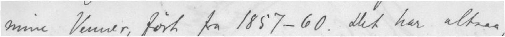mine Venner, først fra 1857 - 60. Det har altsaa,
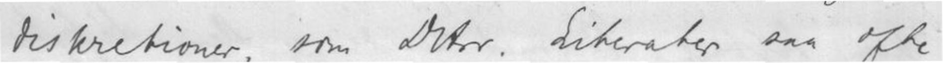diskretioner, som DHrr. Liberater, saa ofte
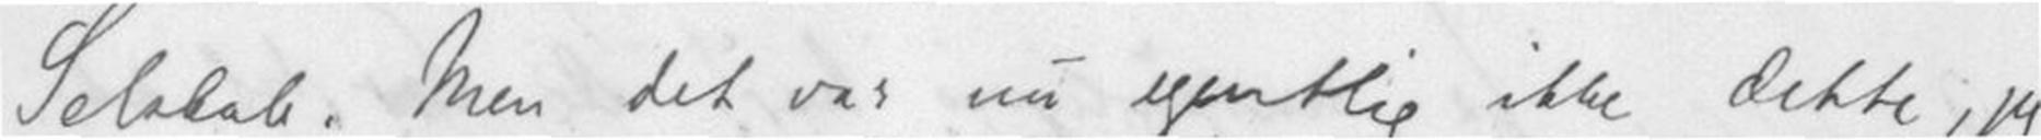Selskab. Men det var nu egentlig ikke dette, jeg
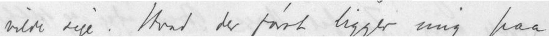vilde sige. Hvad der først ligger mig paa
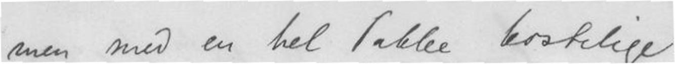men med en hel Pakke kostelige
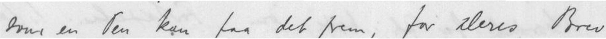som en ven kan faa det frem, for Deres Brev
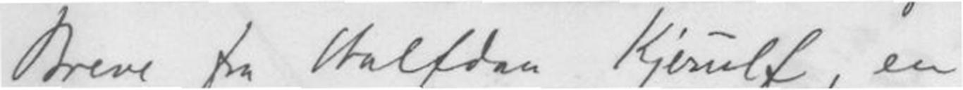Brev fra Halfdan Kjersulf, en
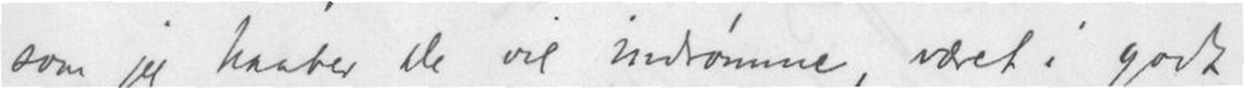som jeg haaber De vil indrømme, været i godt
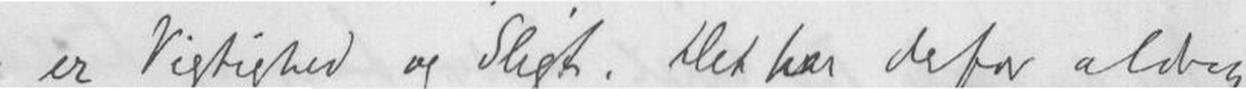er Vigtighed og Sligt. Det har derfor aldrig
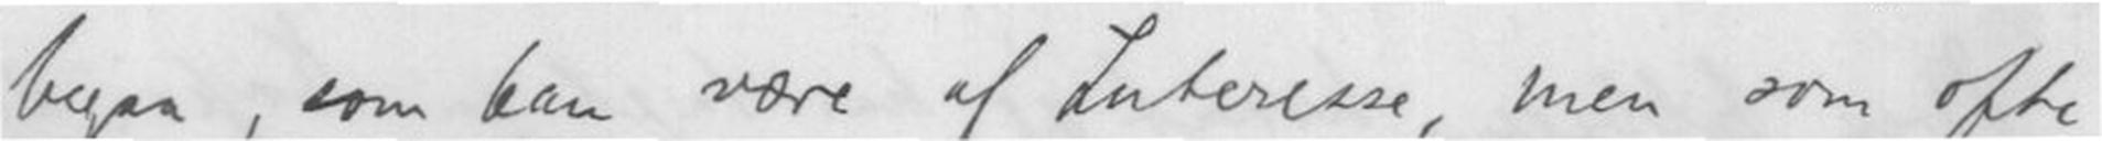begaa, som kan være af Interesse, men som ofte
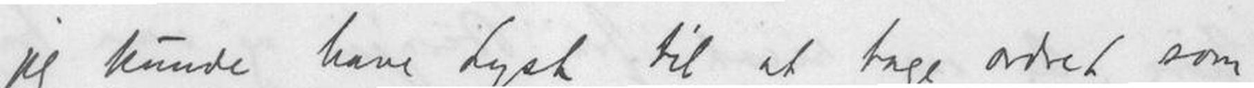jeg kunde have Lyst til at tage ordet som
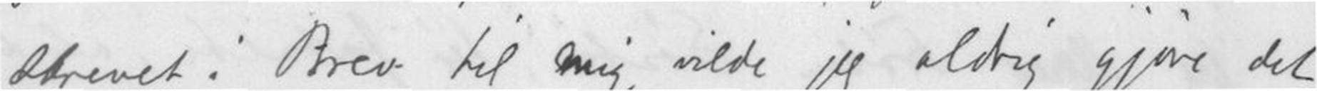skrevet i Brev til mig, vilde jeg aldrig gjøre det
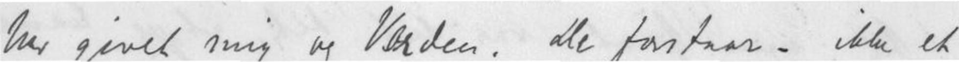har Nu gjevet mig og Verden. De forstaar - ikke et
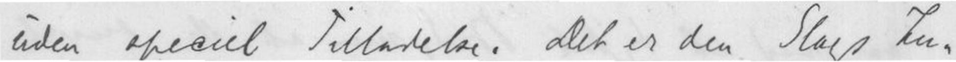uden spesiel Tilladelse. Det er den Slags In=,
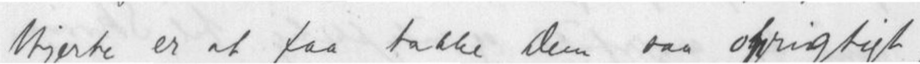Hjerte er at faa takke Dem saa oprigtigt,
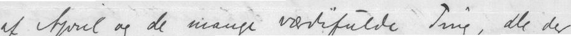af April og de mange værdifulde Ting, De der
